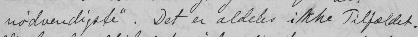nødvendigste". Det er aldeles ikke Tilfældet.
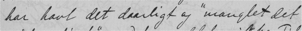har havt det daarligt og "manglet det
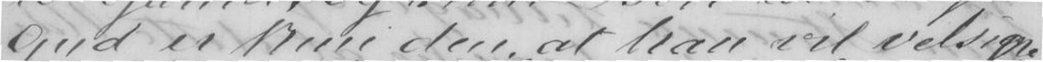Gud er kun, at han vil velsigne
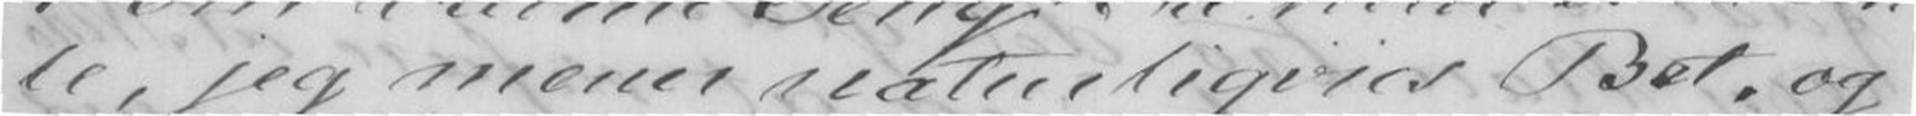le, jeg mener naturligviis Bet. og
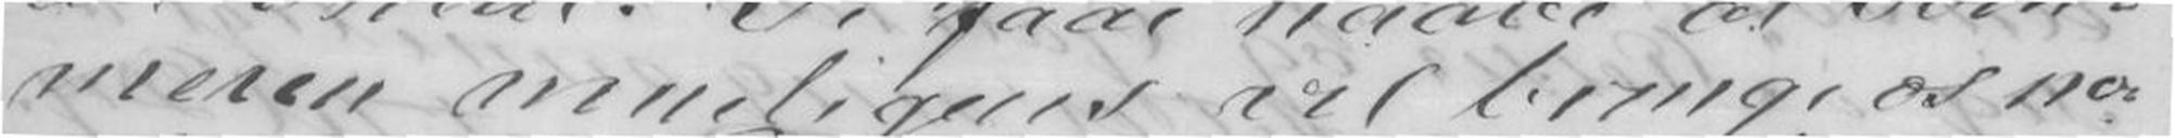meren muligens vil bringe os no-
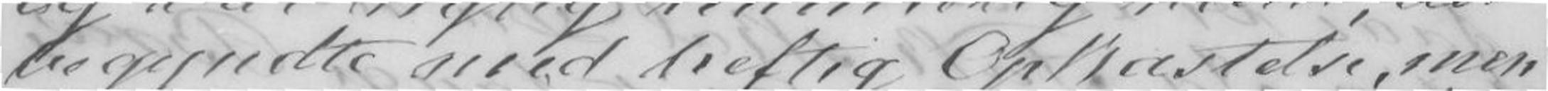begyndte med egen heftig Opkastelse, men
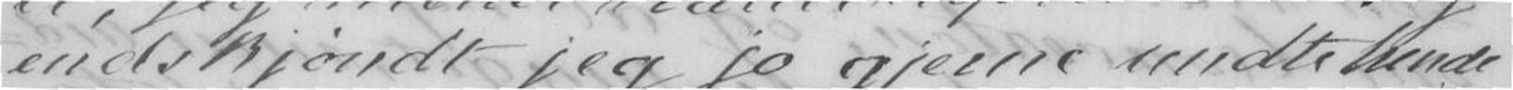endskjøndt jeg jo gjerne undte hende
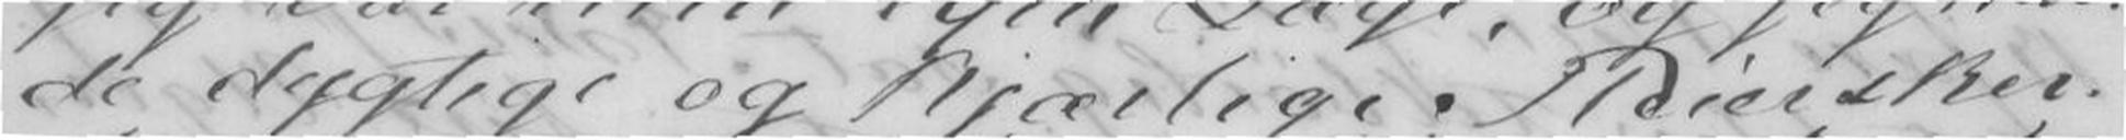de dygtige og kjærlige Pleiersker.
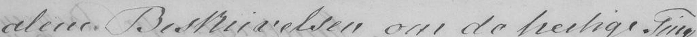alene Beskrivelsen om de herlige Ting
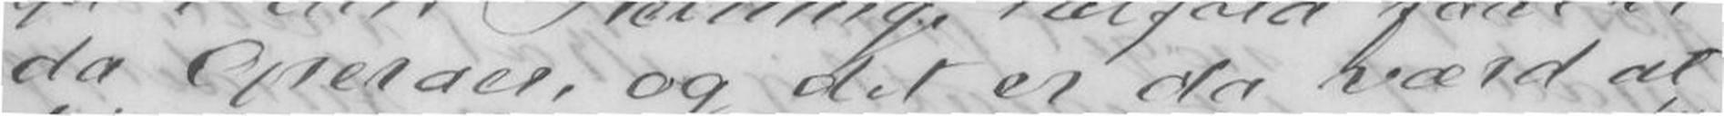da Operaer, og det er da værd at
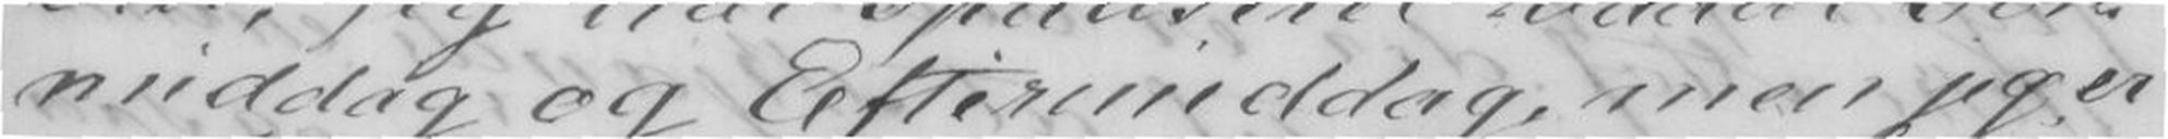middag og Eftermiddag, men jeg er
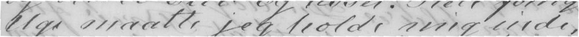Uge maatte jeg holde mig inde,
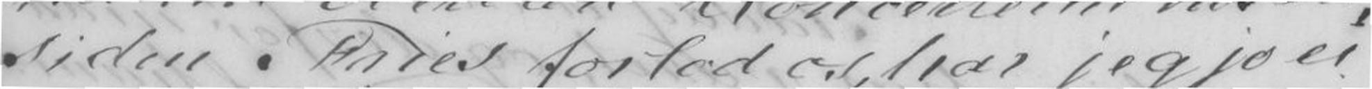siden Fries forlod os, har jeg jo ei
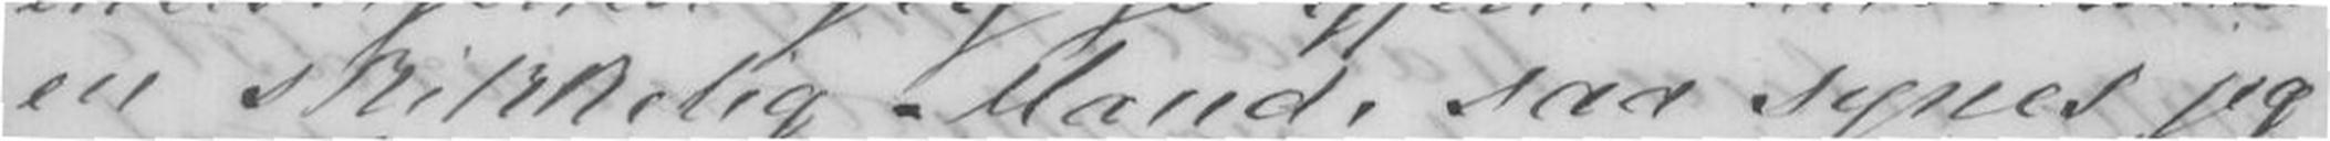en Skikkelig Mand, saa synes jeg
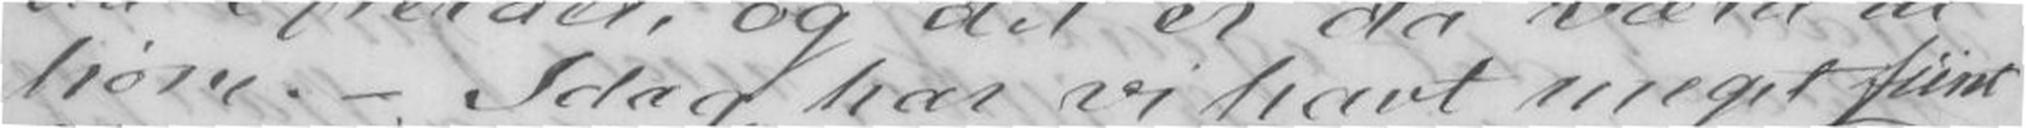høre. - Idag har vi havt meget fiint
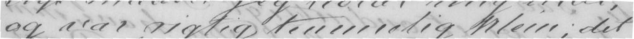og var rigtig temmelig klein; det
